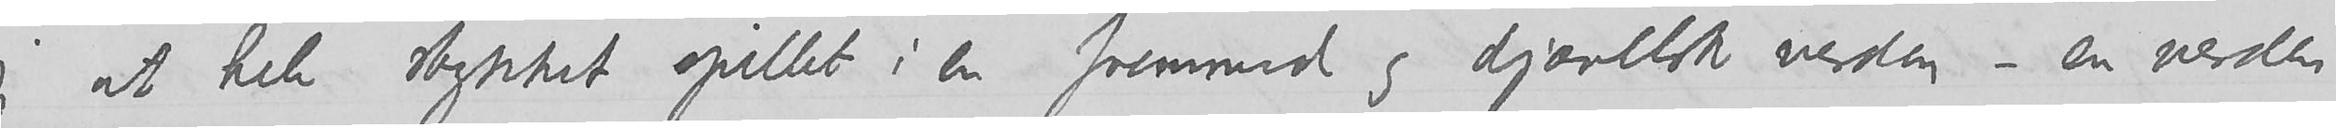jeg at hele stykket spillet i en fremmed og djævelsk verden – en verden
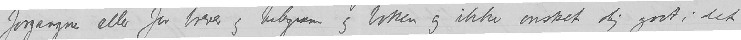forgangne eller for brever og telegram og boken og ikke ønsket dig godt i det
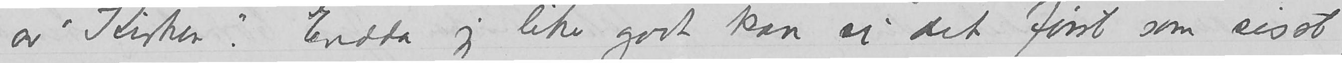av «Kirken». Endda jeg like godt kan si det først som sisst,
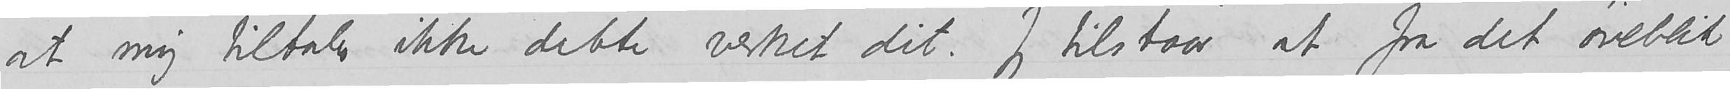at mig tiltaler ikke dette verket dit.  Jeg tilstaar at fra det øieblik
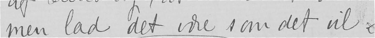men lad det være som det vil.
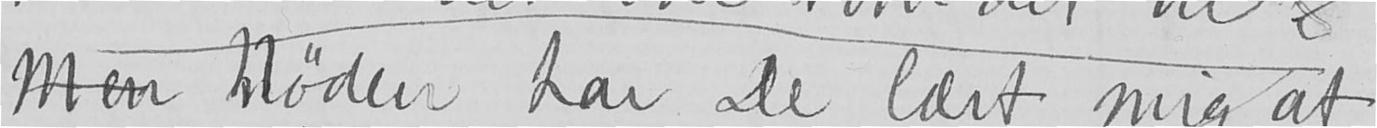Men Nøden har De lært mig at
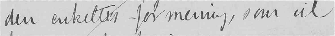den enkeltes formening, som vil
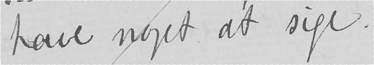have noget at sige.
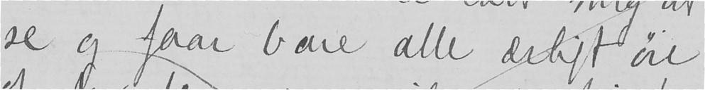se og faar bare alle ærligt øie
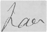paa
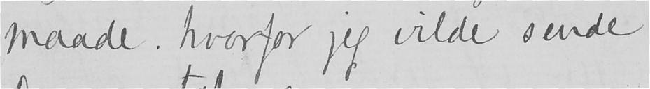maade. hvorfor jeg vilde sende
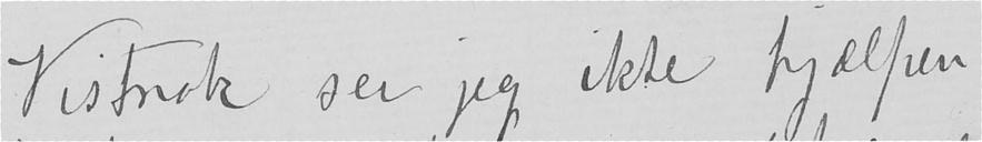Vistnok ser jeg ikke hjælpen
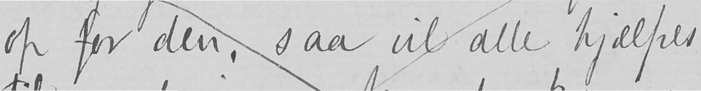op for den, saa vil alle hjælpes
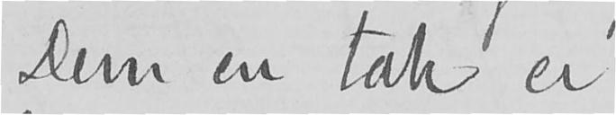Dem en tak er
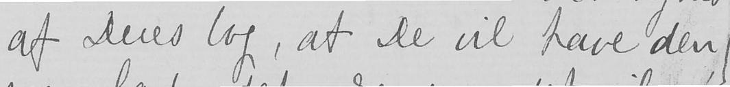af Deres bog, at De vil have den,
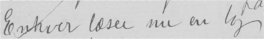Enhver læser nu en bog
